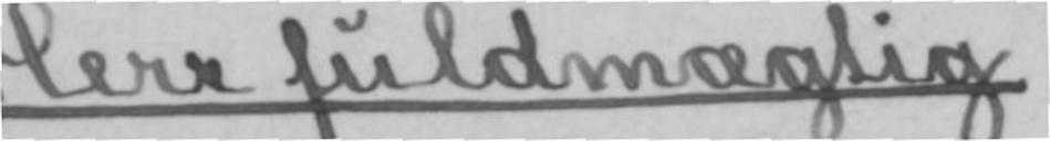Herr fuldmægtig
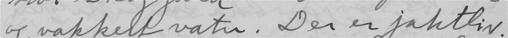og vakkert vatn. Der er jaktliv.
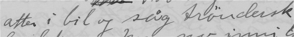atter i bil og såg tröndersk.
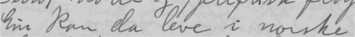Ein kan da leve i norske
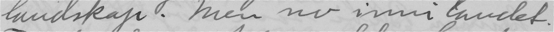landskap. Men no inni landet.
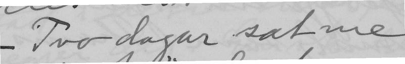Tvo dagar sat me
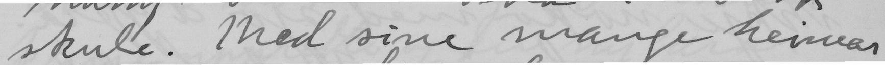skule. Med sine mange heimar
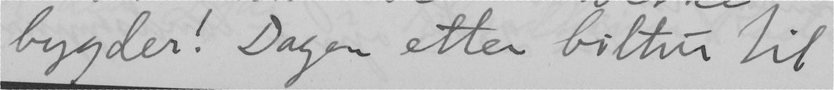bygder! Dagen etter biltur til
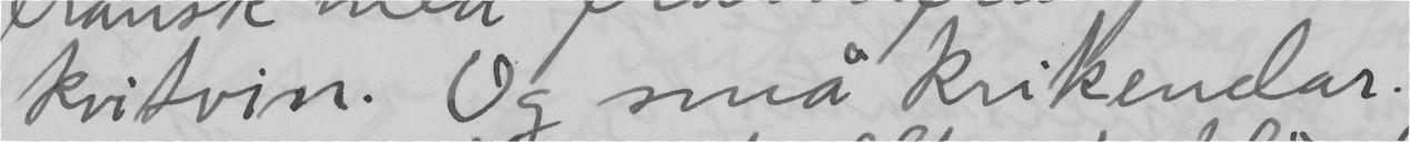kvitvin. Og små krikendar.
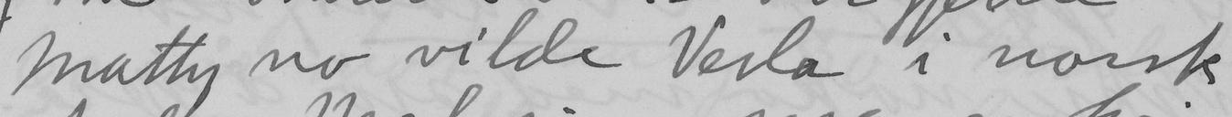Matty no vilde Vesla i norsk
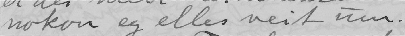nokon eg elles veit um.
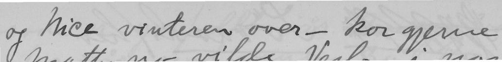og Nice vinteren over – kor gjerne
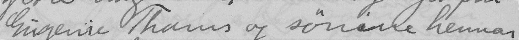Eugenie Thams og sönane hennar
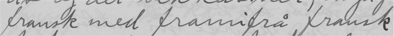fransk med framifrå fransk
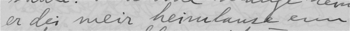er dei meir heimlause enn
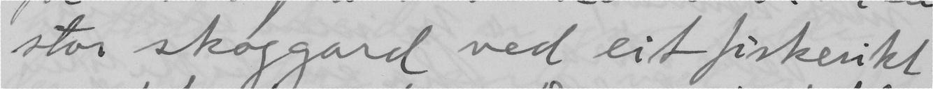stor skoggard ved eit fiskerikt
